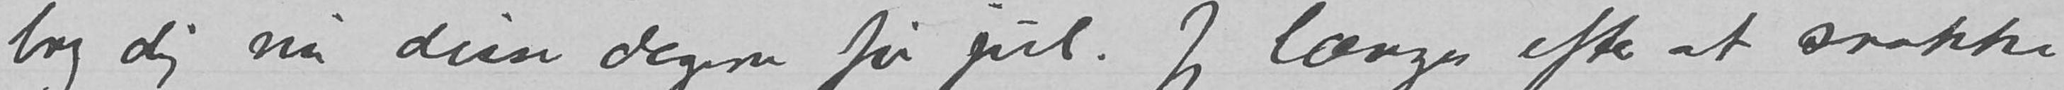bry dig nu disse dagene før jul. Jeg længes efter at snakke
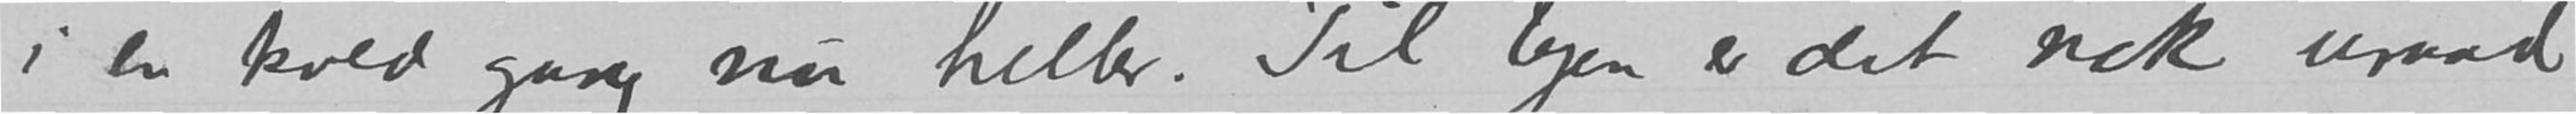i en kold gang nu heller. Til byen er det nok uraad
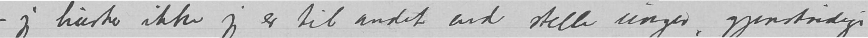jeg husker ikke jeg er til andet end stelle unger, gjenstridige
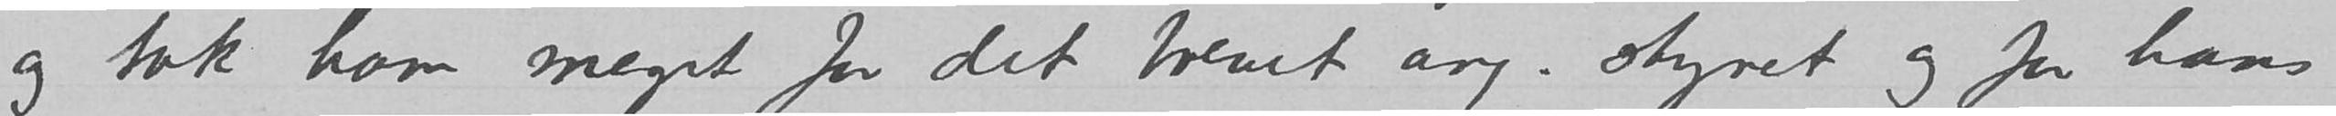og tak ham meget for det brevet ang. styret og for hans
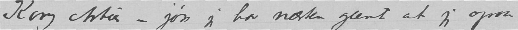Kong Artur – jøss jeg har næsten glemt at jeg ogsaa
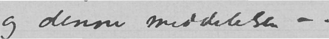og denne meddelelse –.
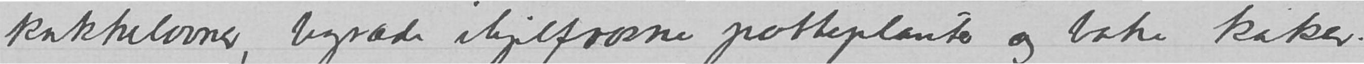kakkelovner, begræde ihjelfrosne potteplanter og bake kaker.
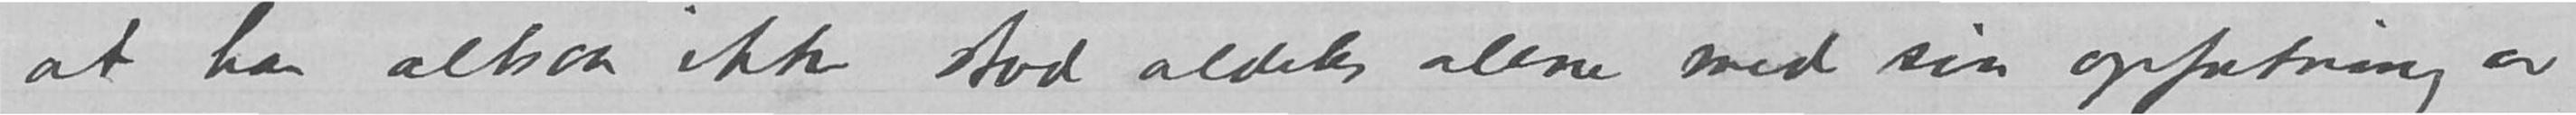at han altsaa ikke stod aldeles alene med sin opfatning av
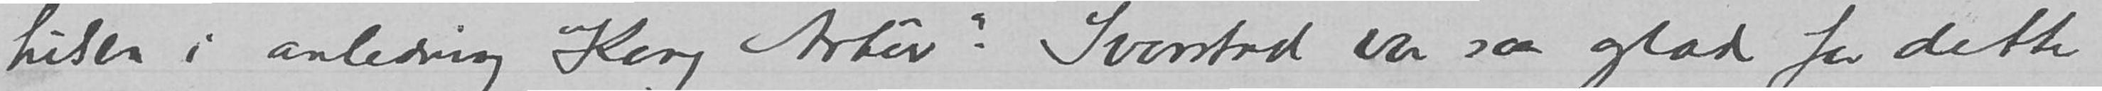hilsen i anledning Kong Artur! Svarstad var saa glad for dette
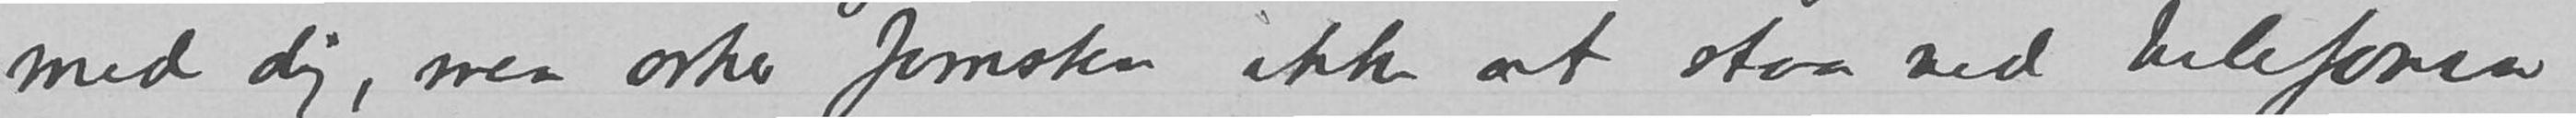med dig, men orker forresten ikke at staa med telefonen
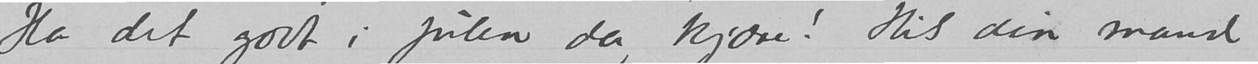Ha det godt i julen da, kjære! Hils din mand
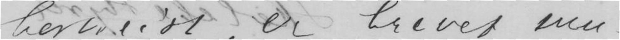bortreist, er brevet mu-
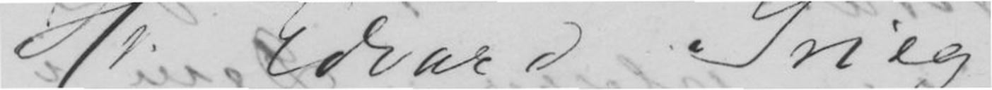Hr Edvard Grieg.
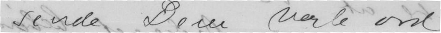sende Dem nogle ord
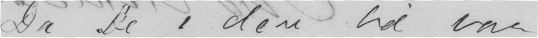Da De i den tid var
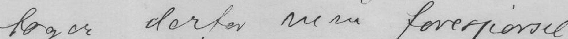tager derfor min forespørgsel.
